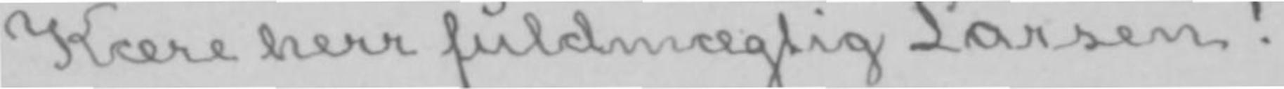Kære herr fuldmægtig Larsen!
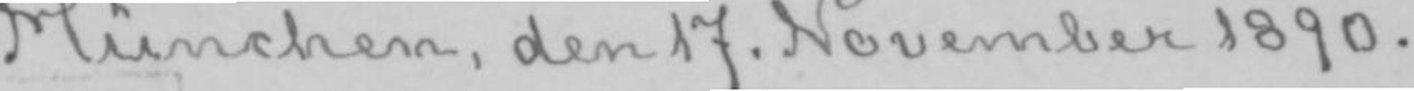München, den 17. November 1890.
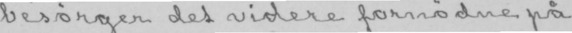besørger det videre fornødne på
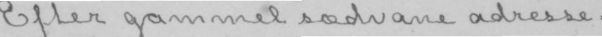Efter gammel sædvane adresse-
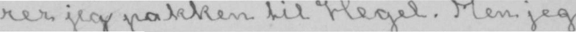rer jeg pakken til Hegel. Men jeg
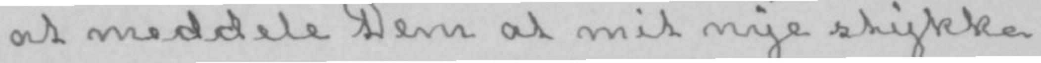at meddele Dem at mit nye stykke
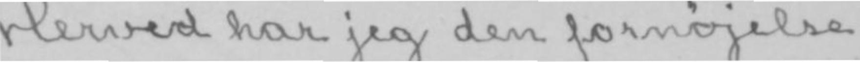Herved har jeg den fornøjelse
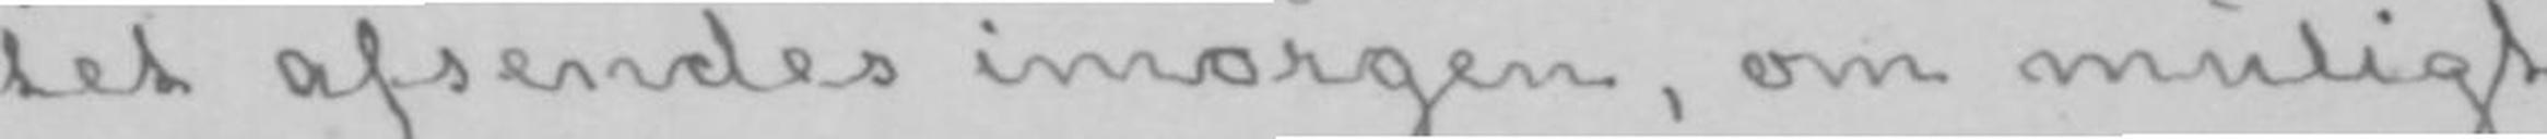tet afsendes imorgen, om muligt
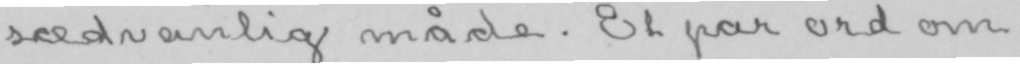sædvanlig måde. Et par ord om
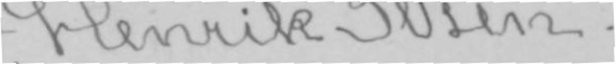Henrik Ibsen.
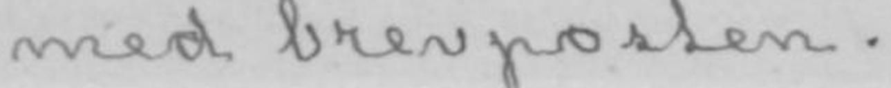med brevposten.
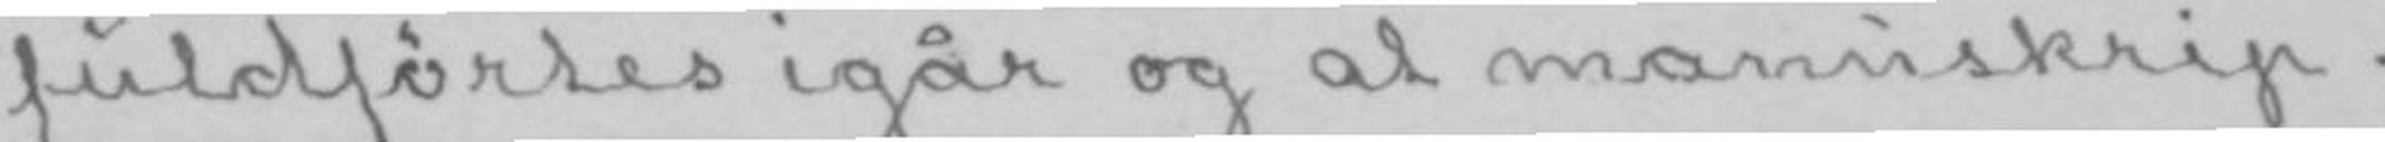fuldførtes igår og at manuskrip-
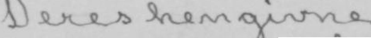Deres hengivne
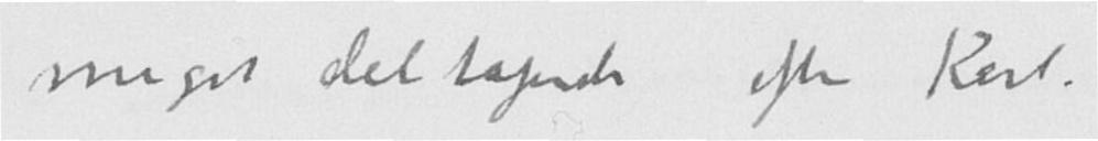meget deltagende efter Karl.
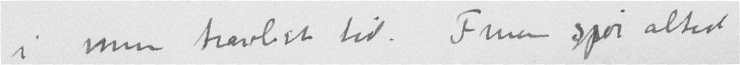i min travleste tid. Fruen spør altid
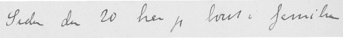Siden den 20 har jeg boet i familien
